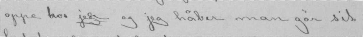oppe hos jer og jeg håber man gør sit
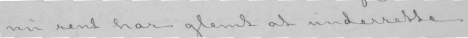nu rent har glemt at underrette
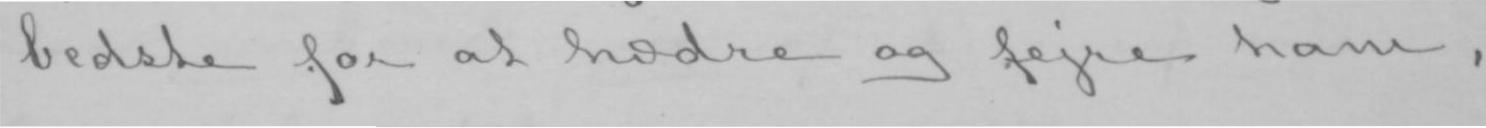bedste for at hædre og fejre ham,
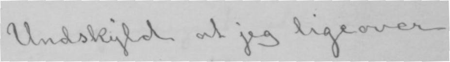Undskyld at jeg ligeover
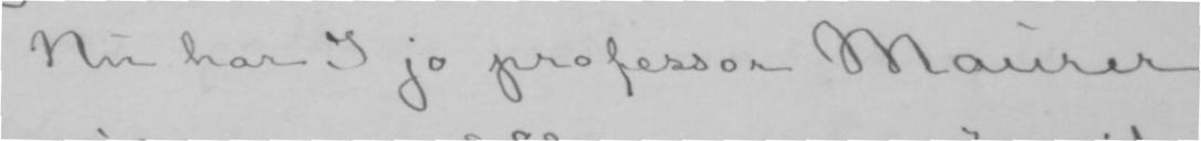Nu har I jo professor Maurer
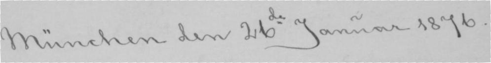München den 26de Januar 1876.
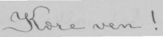Kære ven!
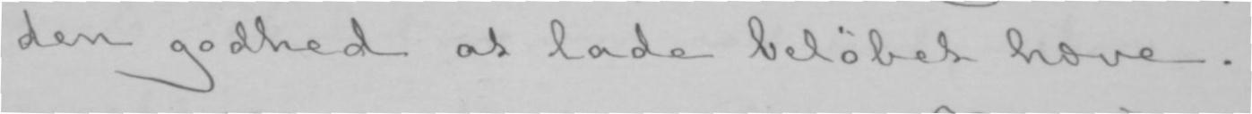den godhed at lade beløbet hæve.-
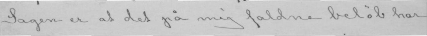Sagen er at det på mig faldne beløb har
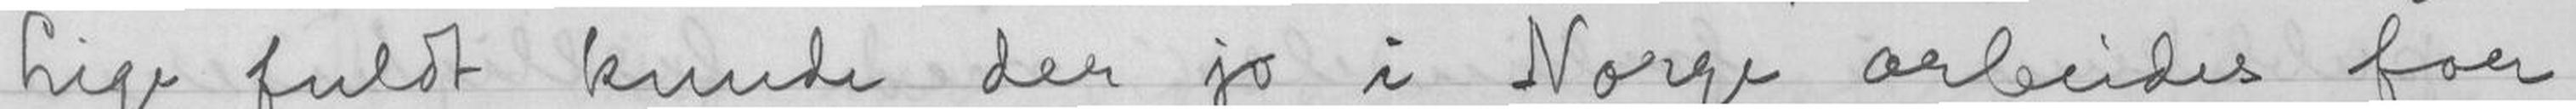Lige fuldt kunde der jo i Norge arbeides for
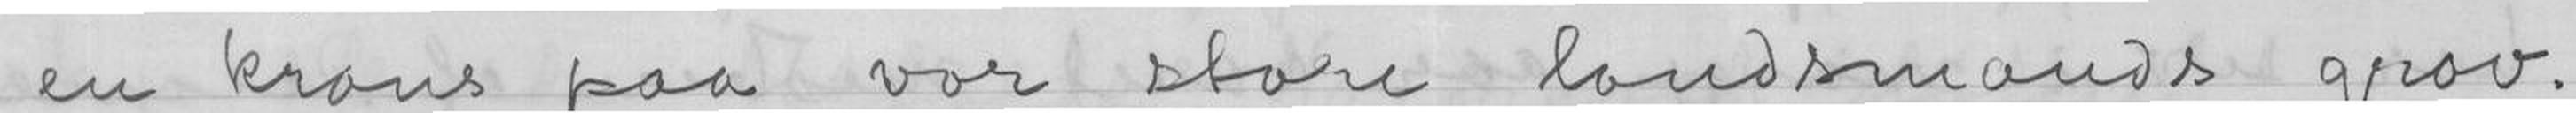en krans paa vor store landsmands grav.
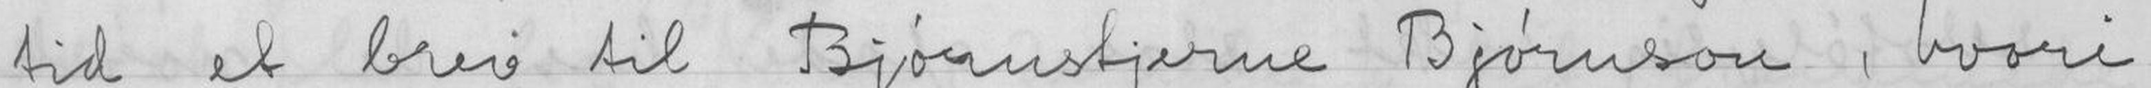tid et brev til Bjørnstjerne Bjørnson, hvori
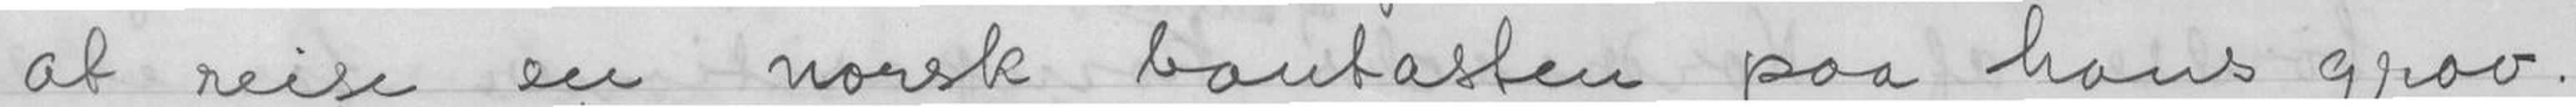at reise en norsk bautasten paa hans grav.
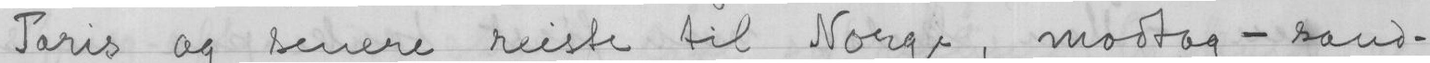Paris og senere reiste til Norge, modtog - sand-
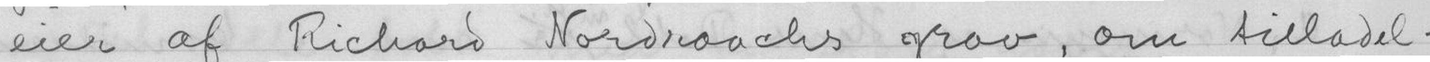eier af Richard Nordraachs grav, om tilladel-
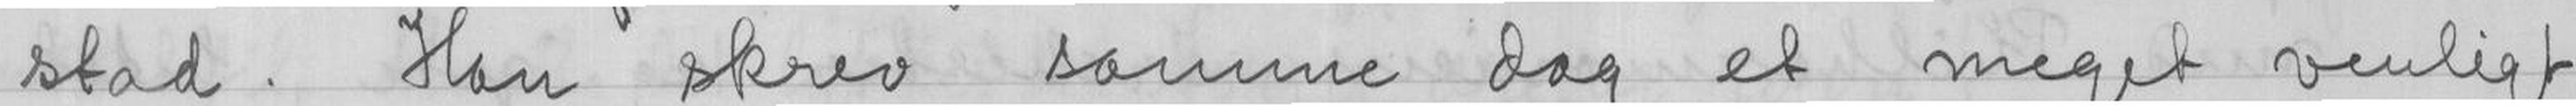stad. Han skrev samme dag et meget venligt
